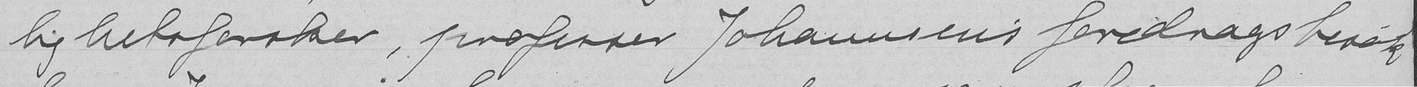lighetsforsker, professer Johannsens foredragsbesøk
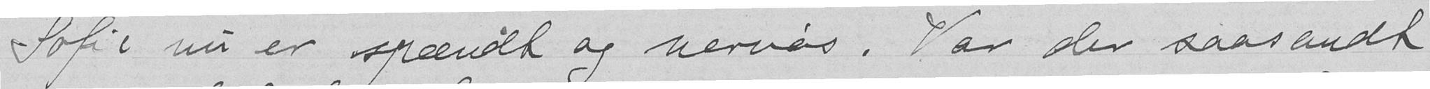-pofie nu er spændt og nervøs. Var der saadandt
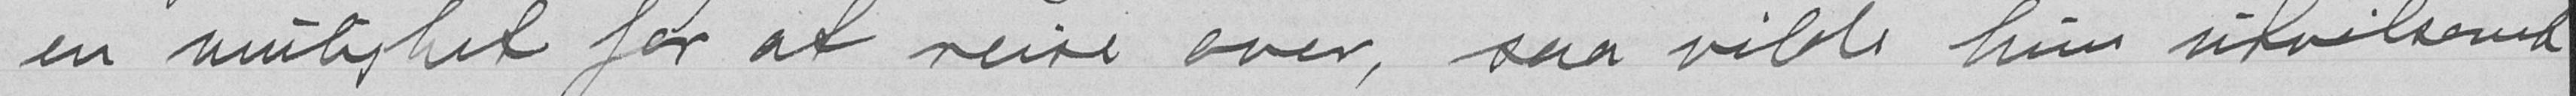en mulighet for at reise over, saa vilde hun utvilsomt
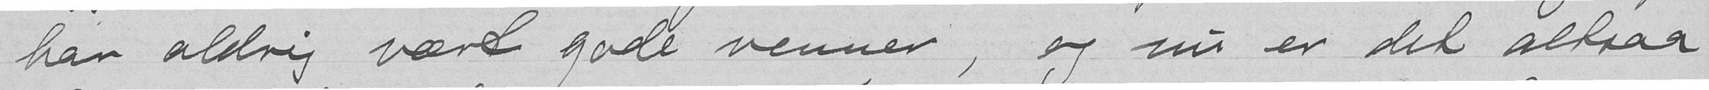han aldrig vært gode venner, og nu er det altsaa
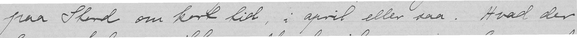paa Stord om kort tid, i april eller saa. Hvad der
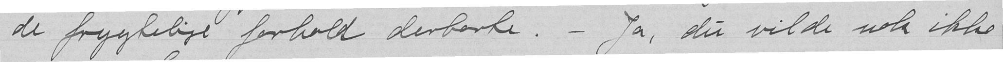de frygtelige forhold derborte. - Ja, du vilde nok ikke
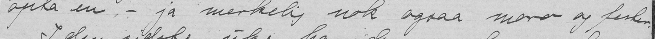opta en ,- ja merkelig nok ogsaa moro og fester.
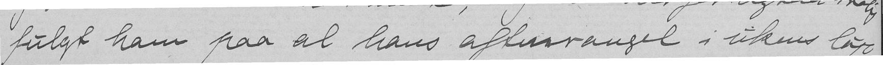fulgt ham paa al hans aftesrangel i ukens løp
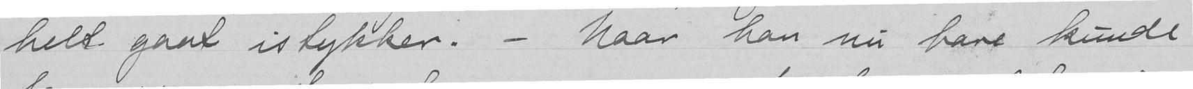helt gaat istykker. - Naar han nu hare kunde
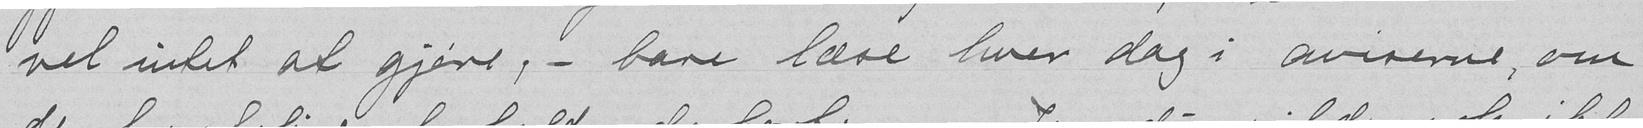vel intet at gjøre, - bare læse hver dag i aviserne, om
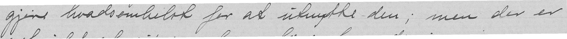gjere hvadsomhelset for at utnytte den; men der er
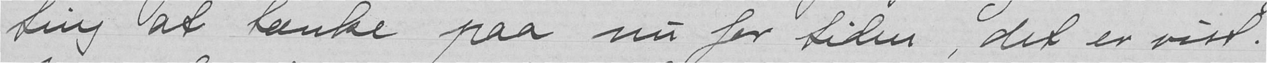ting at tænke paa nu for tiden, det er vist.
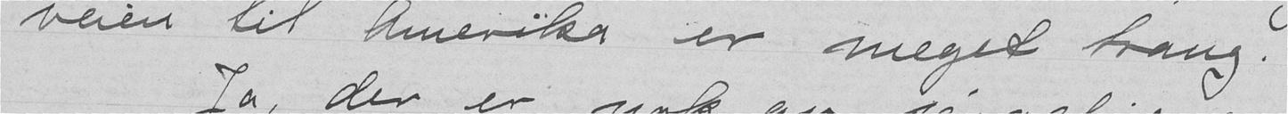veien til Amerika er meget trang.
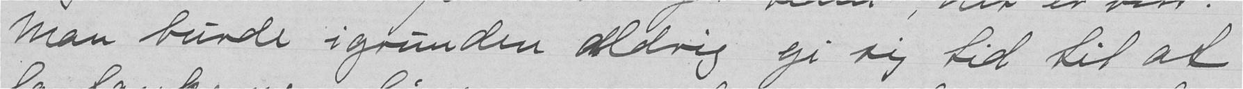Man burde igrunden aldrig gi sig tid til at
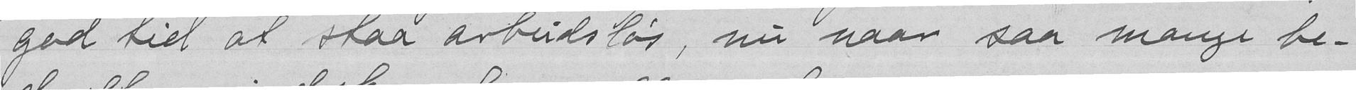god till at staa arbeidsløs, nu naar saa mange be-
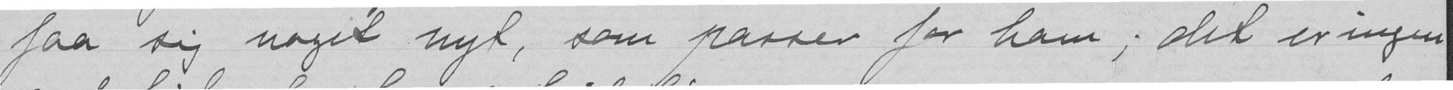faa sig noget nyt, som passer for ham ; det er ingen
